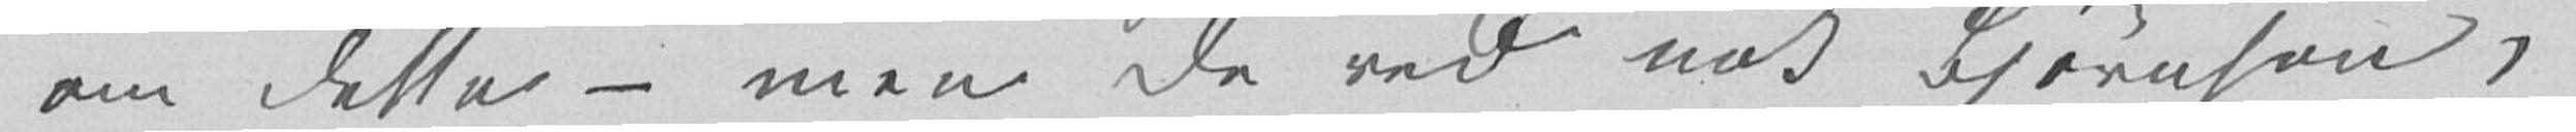om dette – men De ved nok Bjørnson,
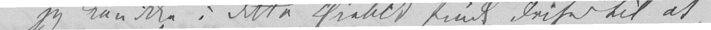jeg kan ikke i dette Øieblik finde Frihed til at
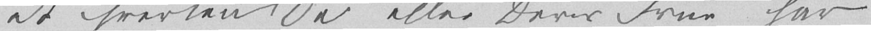at hverken De eller Deres Frue har
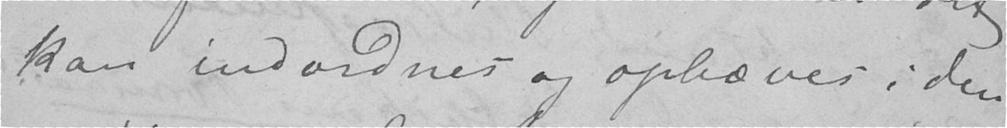kan indordnes og ophæves i den
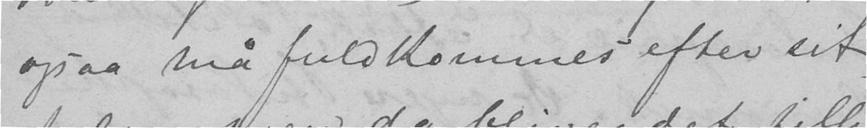ogsaa maa fuldkommes efter sit
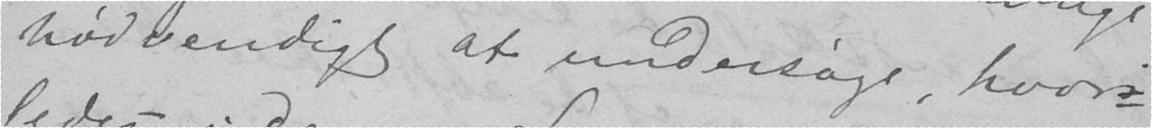nødvendigt at undersøge, hvor-
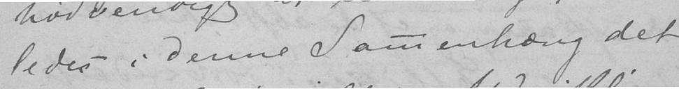ledes i denne Sammenhæng det
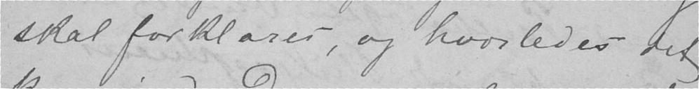skal forklares, og hvorledes det
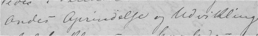Ondes Oprindelse og Udvikling
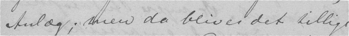Anlæg; men da bliver det tillige
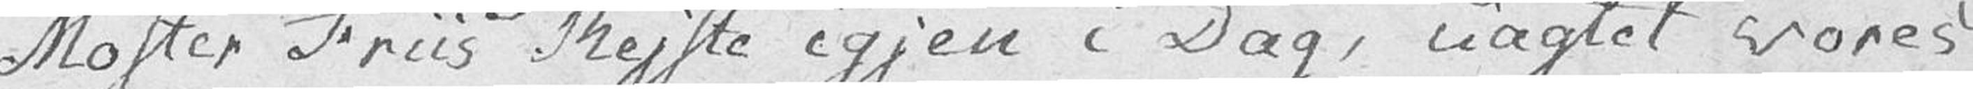Moster Friis Rejste igjen i Dag, uagtet vores
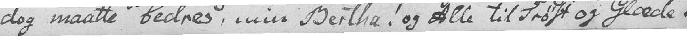dog maatte bedres, min Bertha! og Alle til Trøst og Glæde.
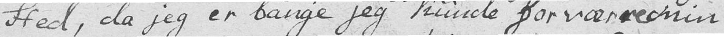Sted, da jeg er bange jeg kunde forværre min
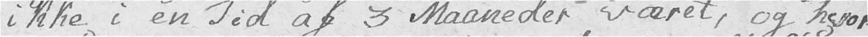ikke i en Tid af 3 Maaneder været, og hvor
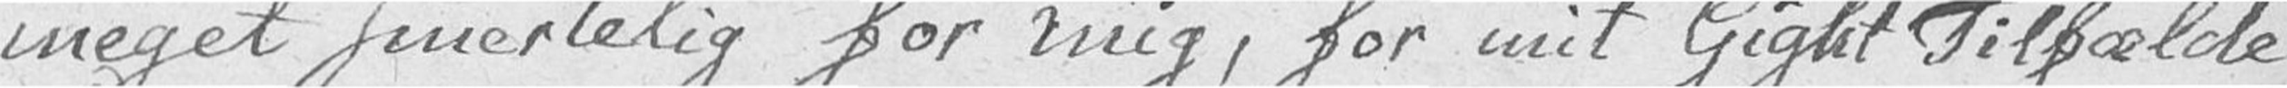meget smertelig for mig, for mit Gight Tilfælde
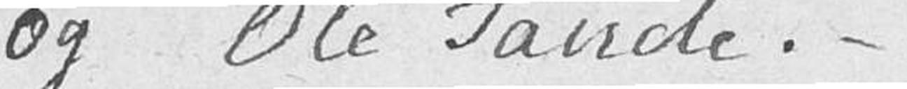og Ole Tande. –
